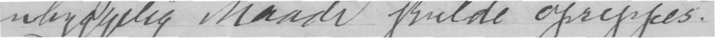uhyggelig Maade skulde oprippes.
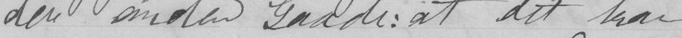den anden Gaade: at et har
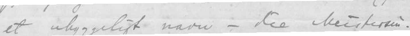et uhyggeligt navn - die Meistersin-
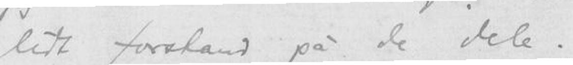lidt forstand på de dele.
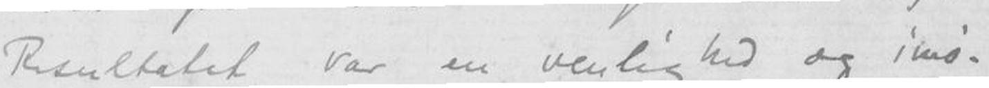Resultatet var en venlighed og imø-
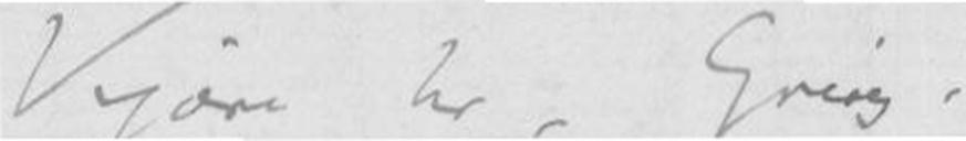Kjære hr. Grieg!
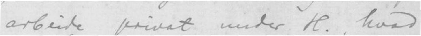arbeide privat under H., hvad
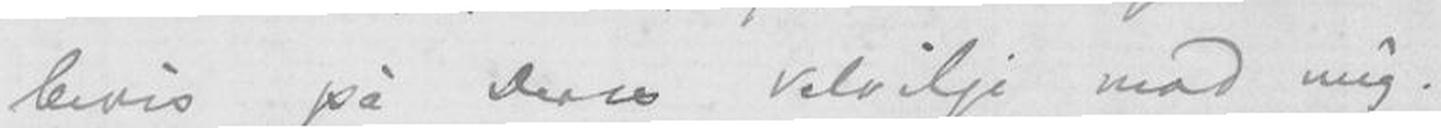bevis på Deres velvilje mod mig.
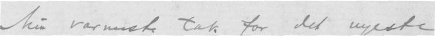Min varmeste tak for det nyeste
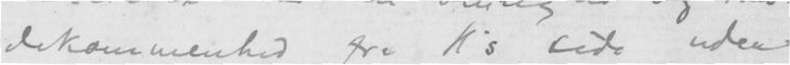dekommenhed fra H's side uden
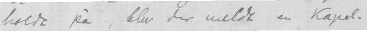holdt på, blev der meldt en kapel-
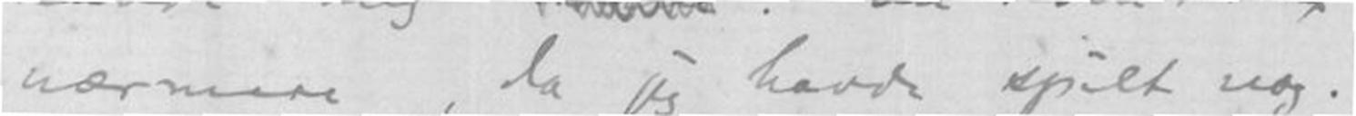nærmere, da jeg havde spilt nog-
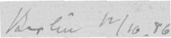Berlin 12/10.86
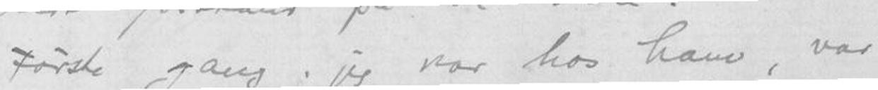Første gang jeg var hos ham, var
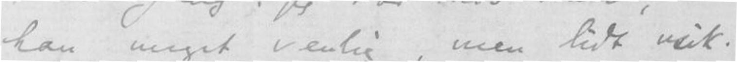han meget venlig, men lidt usik-
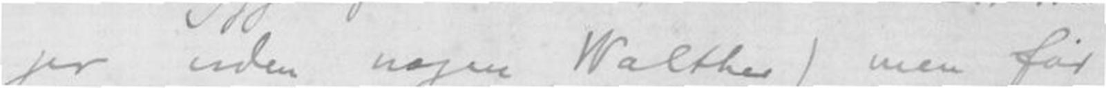ger uden nogen Walther) man får
